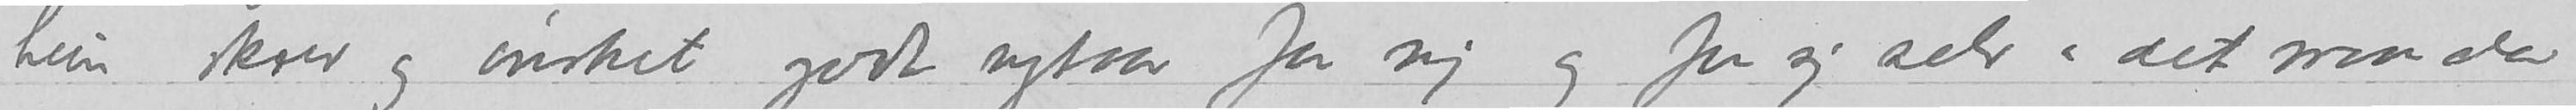hun skrev og ønsket godt nytaar for mig og for sig selv «det maa
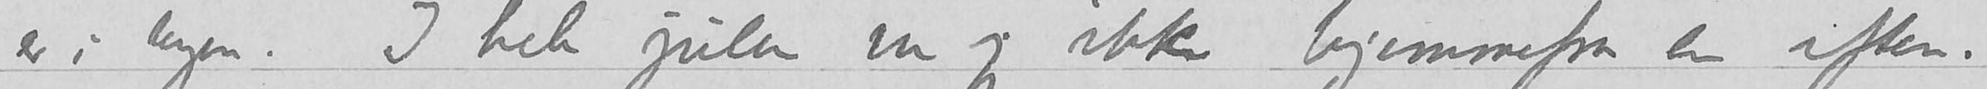er i byen. I hele julen var jeg ikke hjemmefra en aften.
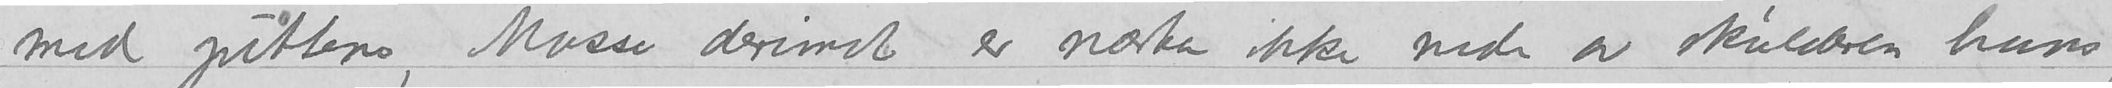med guttene, Mosse derimot er næsten ikke nede av skulderen hans,
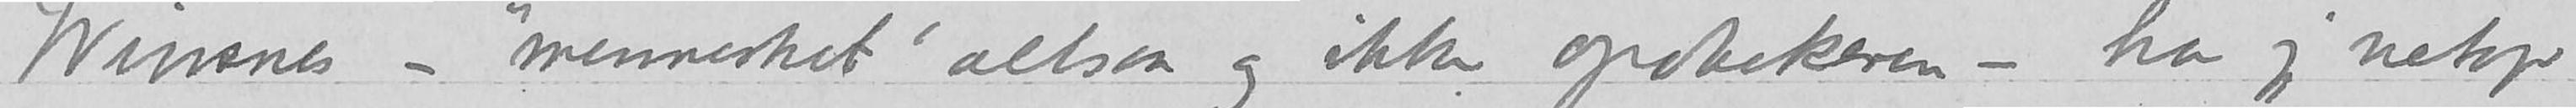Winsnes – «mennesket» altsaa og ikke apotekeren – har jeg netop
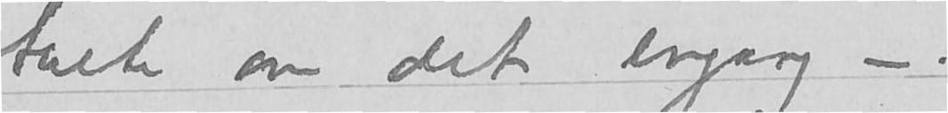talte om det engang –
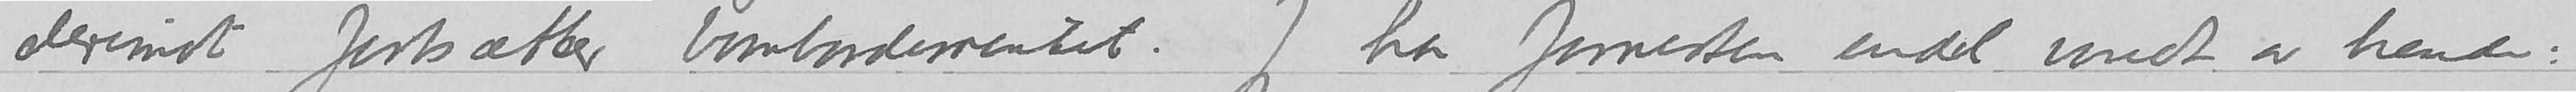Barbra derimot fortsætter bombardementet.  Jeg har forresten en del vondt av hende:
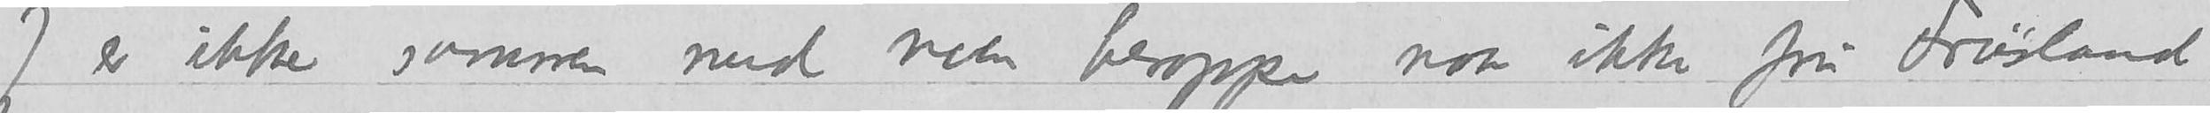Jeg er ikke sammen med noen heroppe naar ikke fru Frøisland
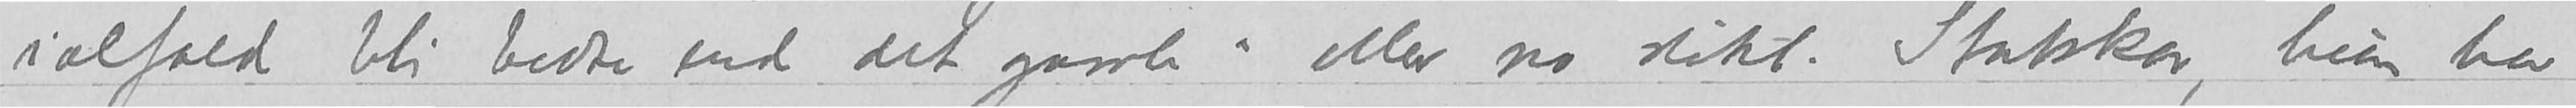da ialfald bli bedre end det gamle» eller no slikt.  Stakkar, hun har
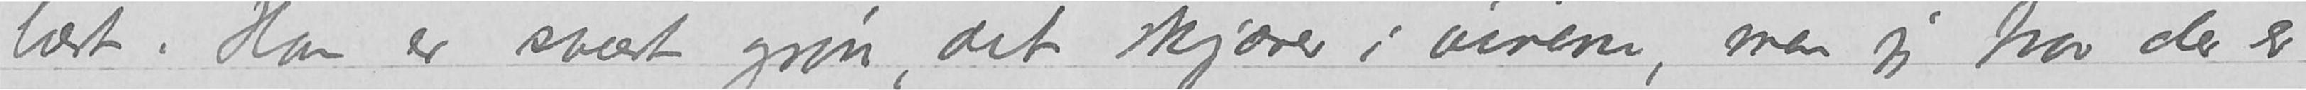læst.  Han er svært grøn, det skjærer i øinene, men jeg tror der er
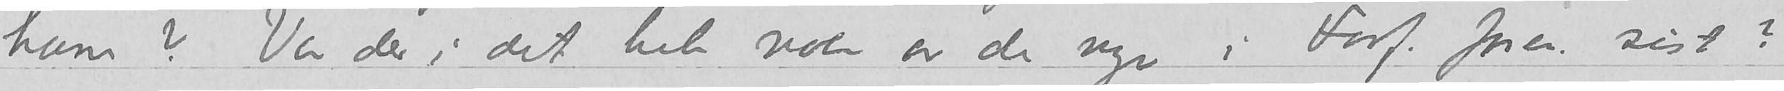ham? Var der i det hele noen av de unge i Forf.foren. sist?
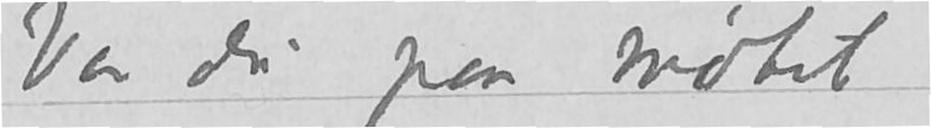Var du på møtet?
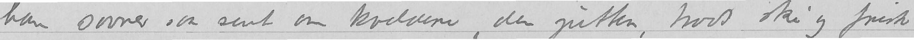han sovner saa sent om kvelden, den gutten, trods ski og frisk
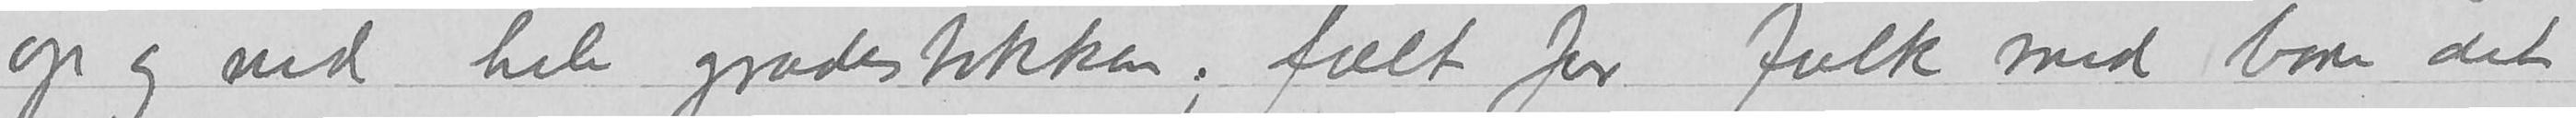op og ned hele gradestokken; fælt for folk med bare det
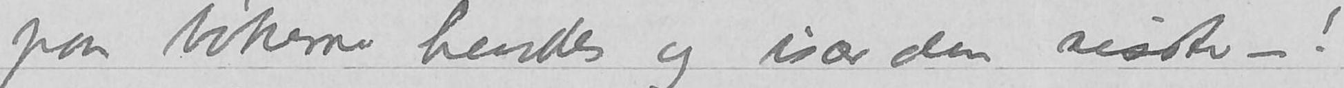paa bøkerne hendes og især den sisste –- !
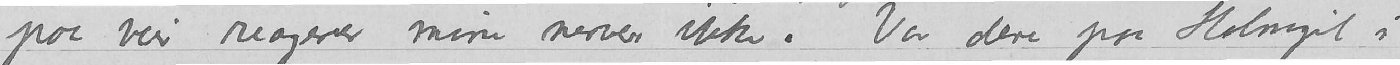paa veir reagerer mine nerver ikke.  Var dere paa Holmgil i
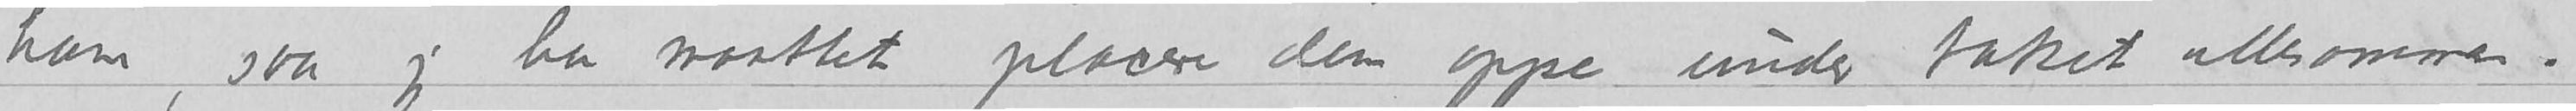ham, saa jeg har maattet placere dem oppe under taket allesammen.
